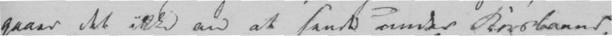gaaer det ikke an at sende under Korsbaand,
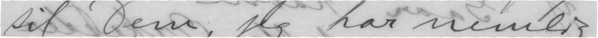til Dem, jeg har nemelig
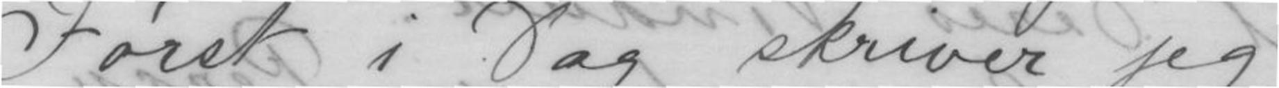Først i Dag skiver jeg
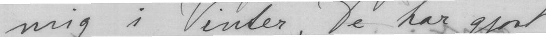mig i Vinter, De har gjort
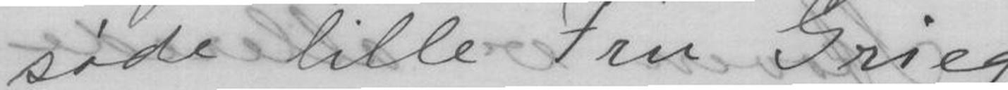søde lille Fru Grieg.
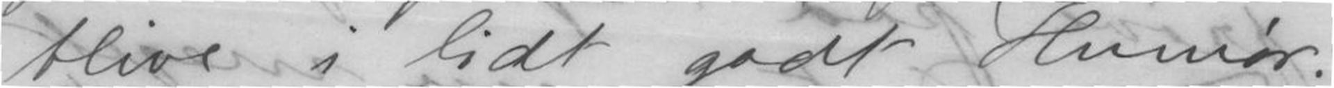blive i lidt godt Humør.
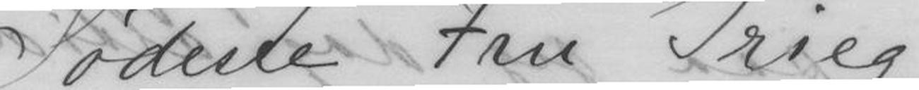Sødeste Fru Grieg
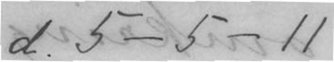d.5-5-11
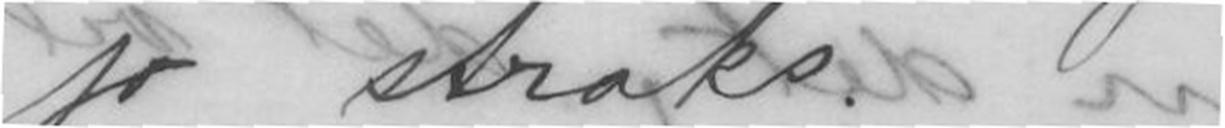jo straks.
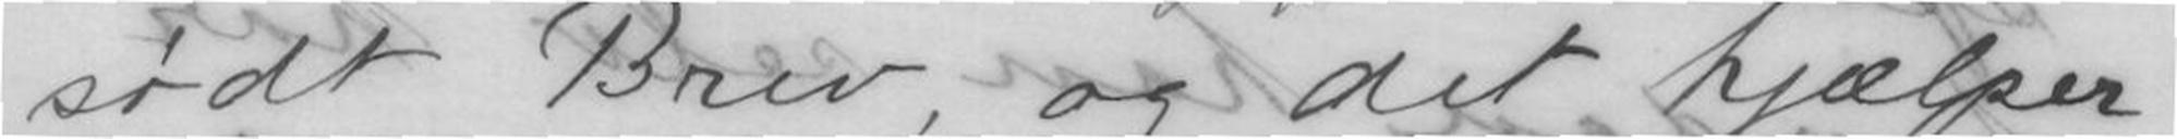sødt Brev, og det hjælper
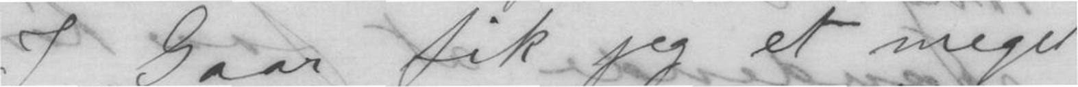I Gaar fik jeg et meget
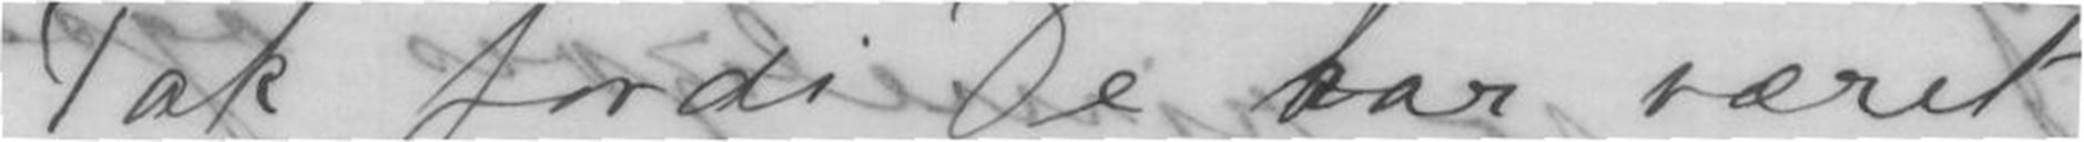Tak fordi De har været
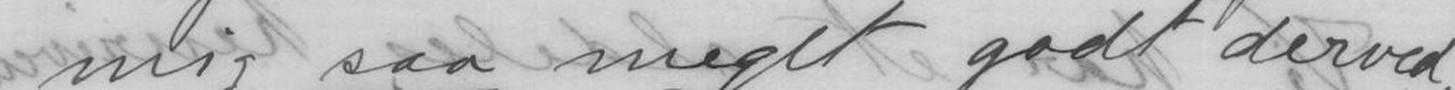mig saa meget godt derved,
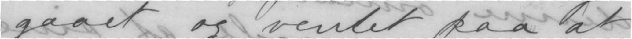gaaet og ventet paa at
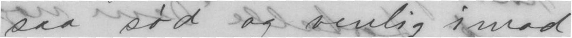saa sød og venlig imod
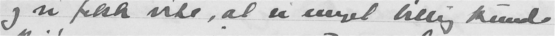og vi fikk vite, at vi meget billig kunde
